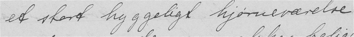et stort hyggeligt hjørneværelse
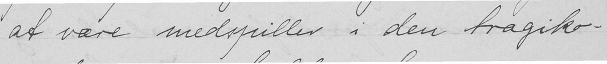at være medspiller i den tragiko-
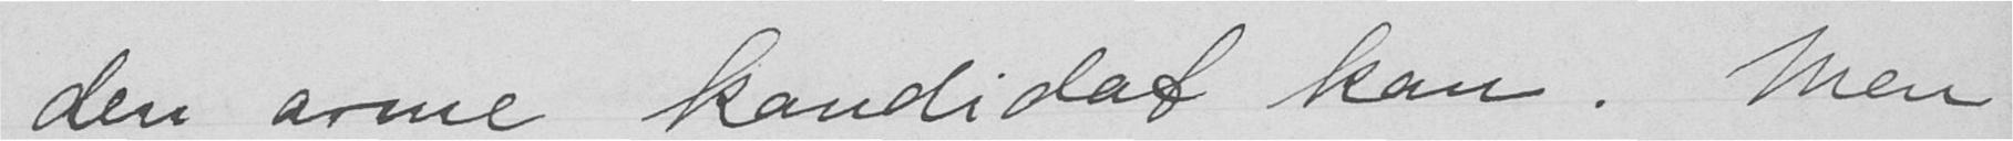den arme kandidat kan. Men
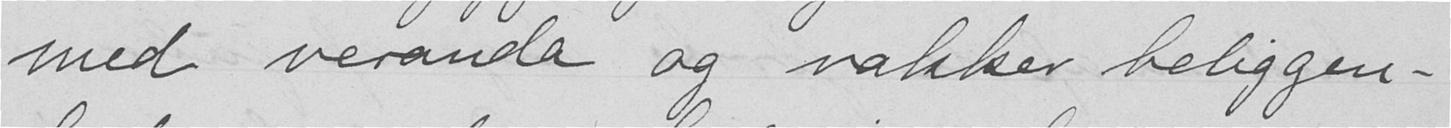med veranda og vakker beliggen-
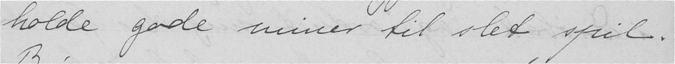holde gode miner til slet spil.
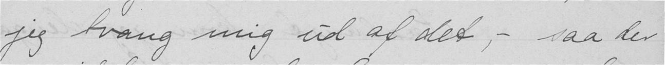jeg tvang mig ud af det, - saa der
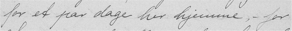for et par dage her hjemme, - for
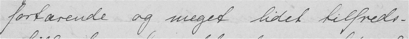fortærende og meget lidet tilfreds-
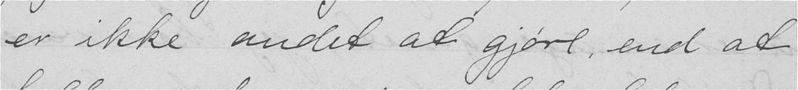er ikke andet at gjøre, end at
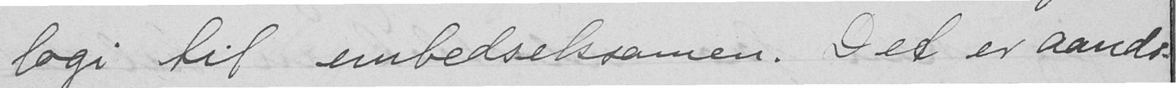logi til embedseksamen. Det er aands-
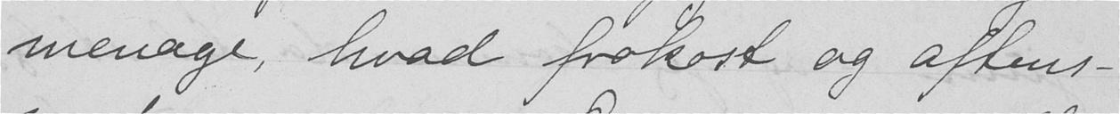menage, hvad frokost og aftens-
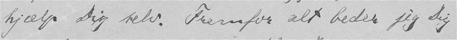hjælp Dig selv. Fremfor alt beder jeg Dig
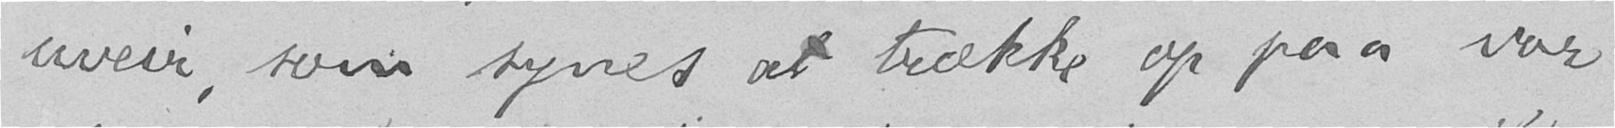uveir, som synes at trække op paa vor
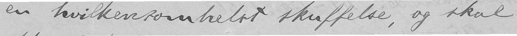en hvilkensomhelst skuffelse, og skal
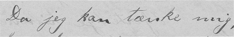Da jeg kan tænke mig,
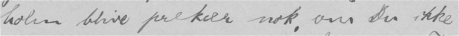holm blive prekær nok, om Du ikke
